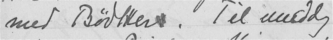med Bødtkers. Til middag
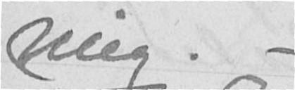mig. -
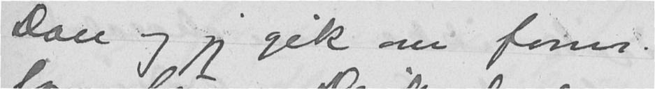Don og jeg gik om form.
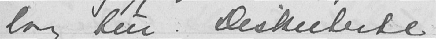lang tur. Diskuterte
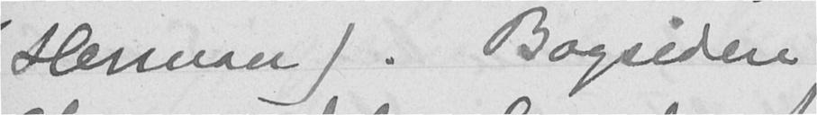Herman). Bagsiden
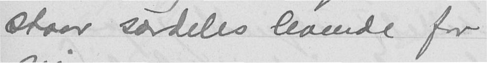staar særdeles levende for
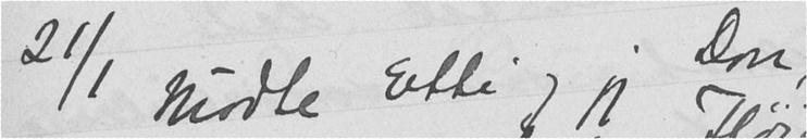21/1 Mødte Etti og jeg Don,
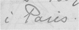i Paris.
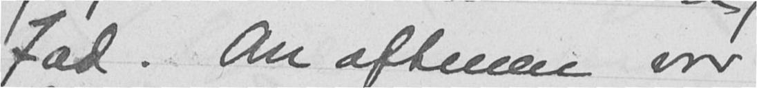fad. Om aftenen var
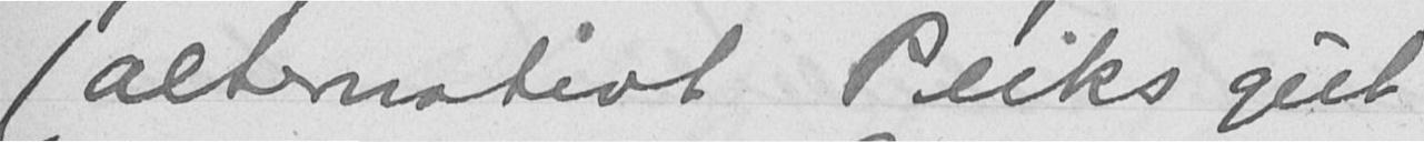(alternativt Peiks gut
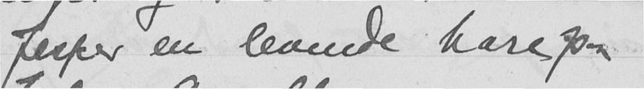Jesper en levende hare paa
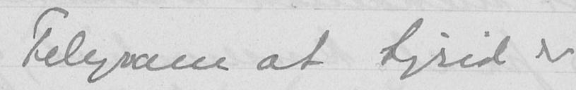Telegram at Sigrid er
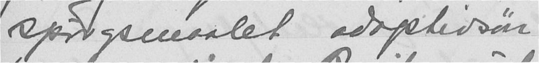spørgsmaalet adoptivsøn
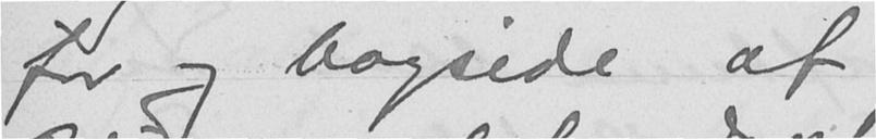for og bagside af
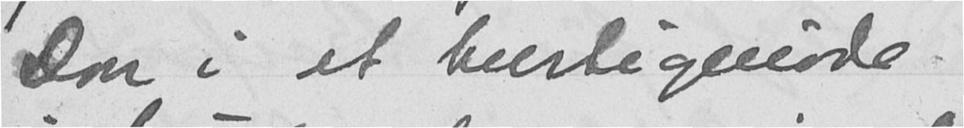Don i et hurtigmøde
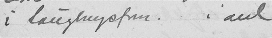i Saugbrugsforn. i anl
